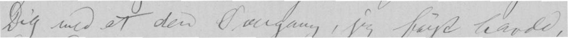Dig med at den Overgang, jeg først havde,
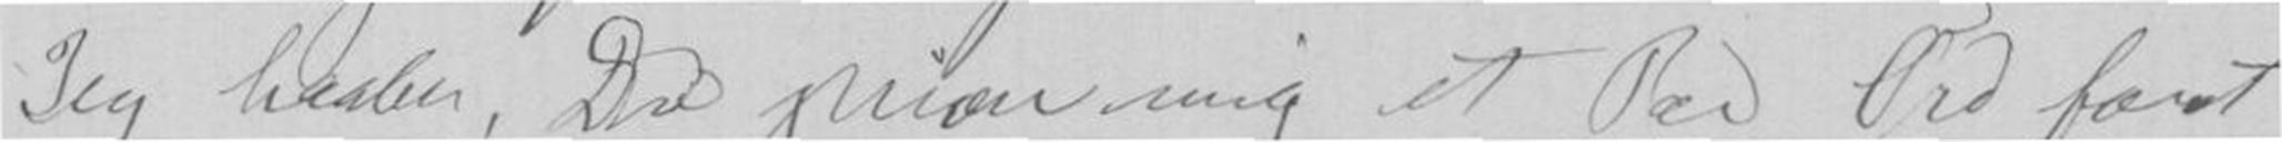Jeg haaber, Du skriver mig et Par Ord forat
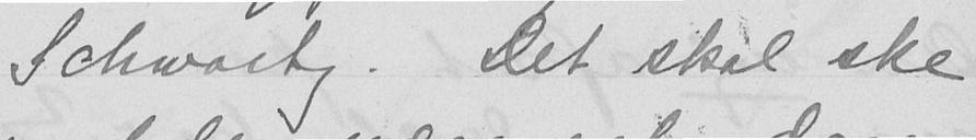Schwartz. Det skal ske
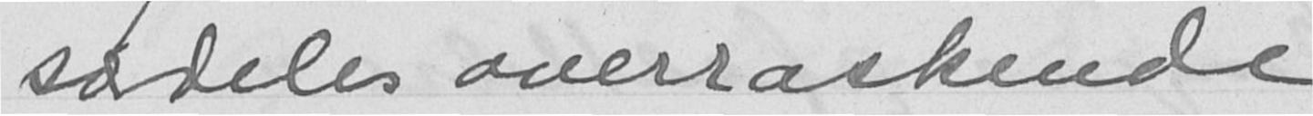særdeles overraskende
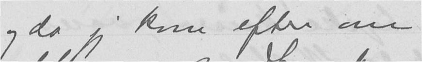og da jeg kom efter om
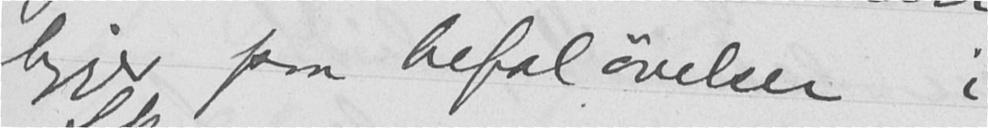ligger paa befaløvelser i
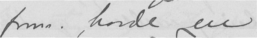form., havde en
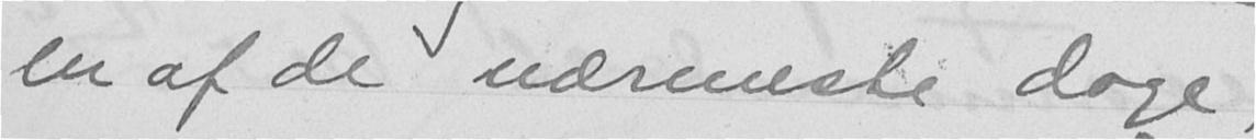en af de nærmeste dage,
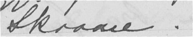Skaane.
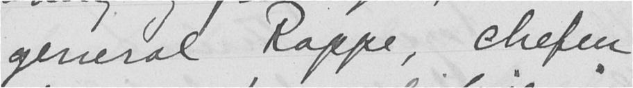general Rappe, chefen
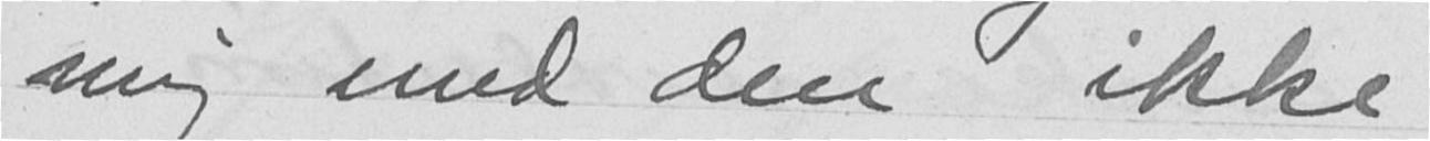mig med den ikke
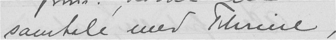samtale med Thrine,
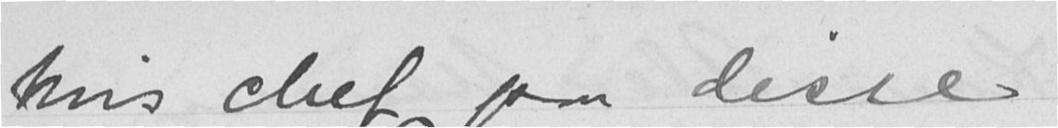hvis chef paa disse
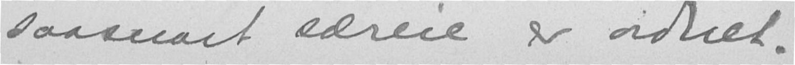saasnart særeie er ordnet.
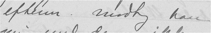efterm. modtog han
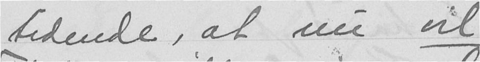tidende, at nu vil
